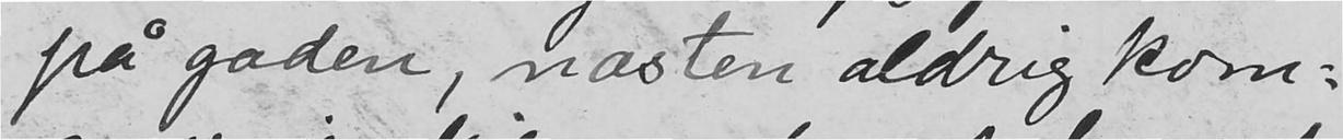på gaden, næsten aldrig kom-
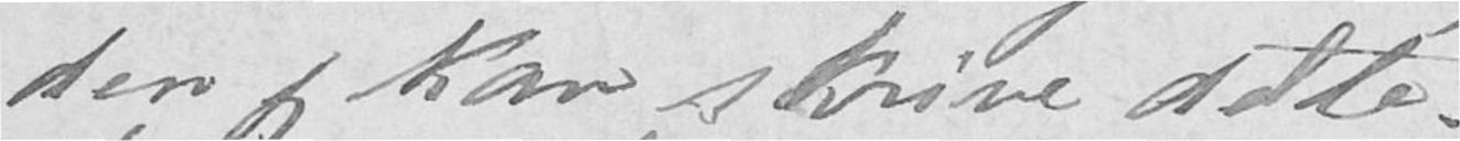den jeg kan skrive dette.
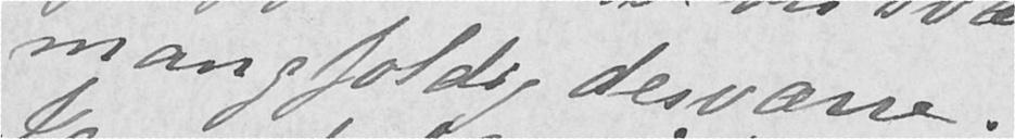mangfoldig desværre.
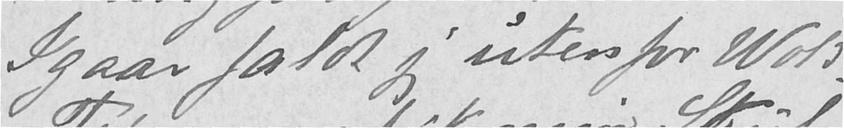Igaar faldt jeg utenfor Wold
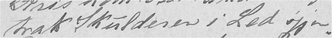trak Skulderen i Led igjen.
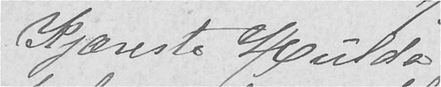Kjæreste Hulda
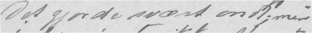Det gjorde svært ondt; men
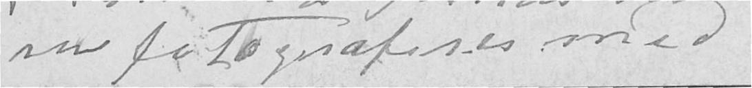ren fotograferes med
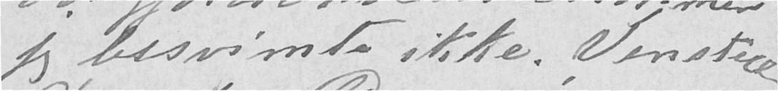jeg besvimte ikke. Venstre
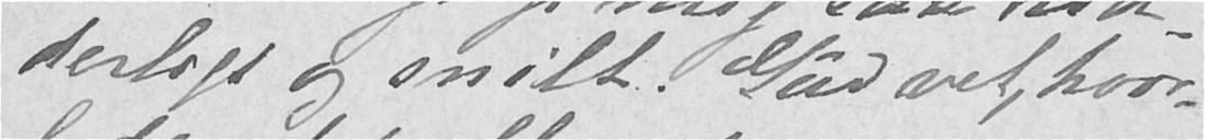derligt og snilt. Gud vet, hvor-
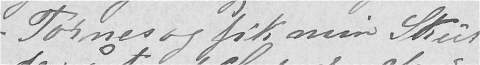-Tornes og fik min Skul-
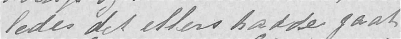ledes det ellers hadde gaat
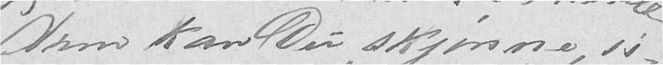Arm kan Du skjønne, si-
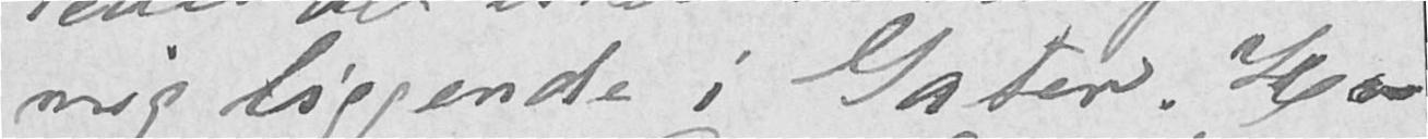mig liggende i Gaten. H
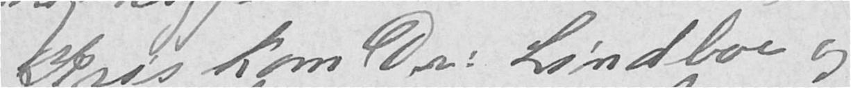Kris kom Dr: Lindboe og
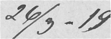26/9-19
